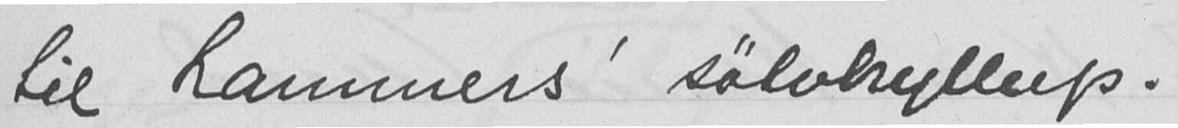til Lammers' sølvbryllup.
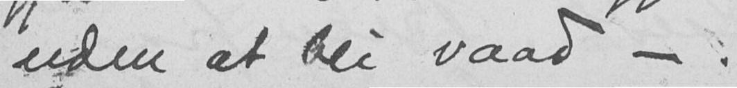uden at bli vaad - .
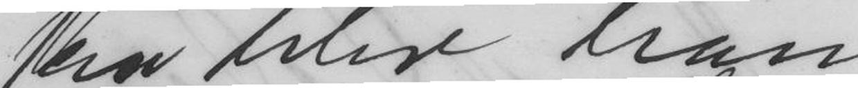saa blev han
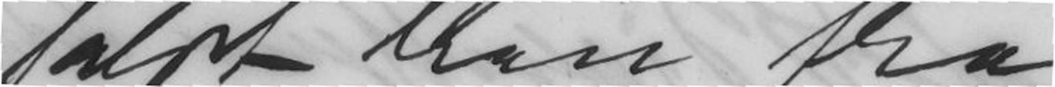faldt han fra.
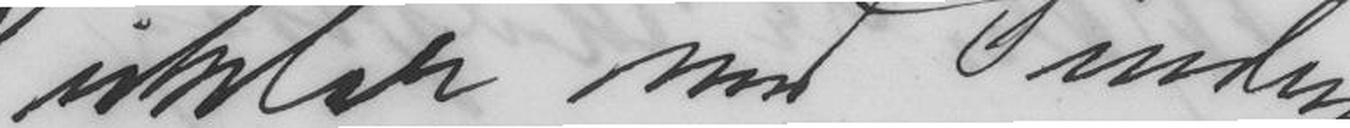uklar med Sinding
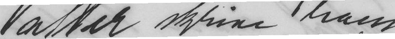atter skrive ham
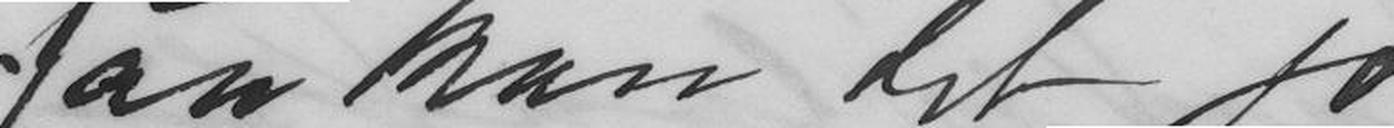saa kan det jo
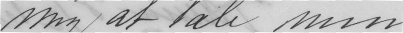mig at tale men
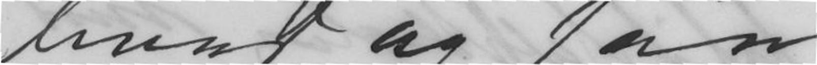hvad og saa
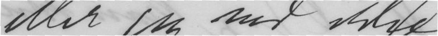eller jeg ved ikke
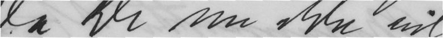Da De nu ikke vil
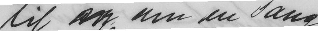til og om en Sang.
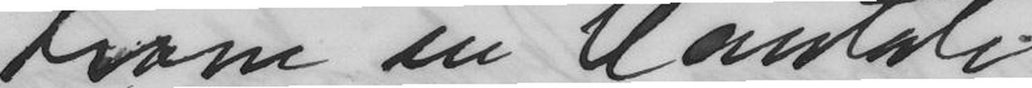have en Cantate
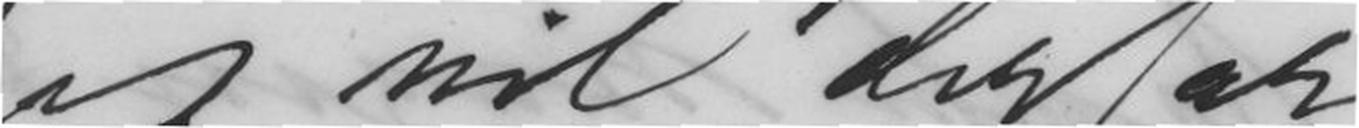Jeg vil derfor
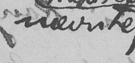nævnte
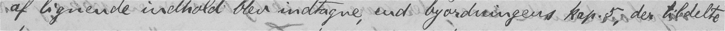af lignende indhold blev indtagne, end byordningens kap. 5, der tildelte
